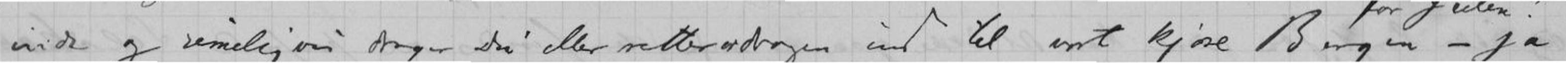inde og rimeligvis drager du eller retter er dragen ind til vort kjære Bergen - ja
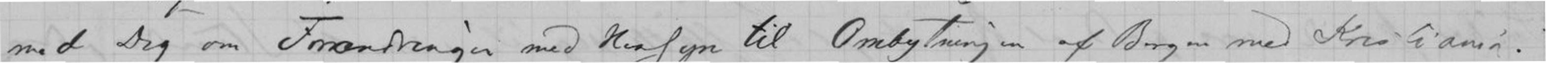med Dig om Forandringen med Hensyn til Ombytningen af Bergen med Kristiania.
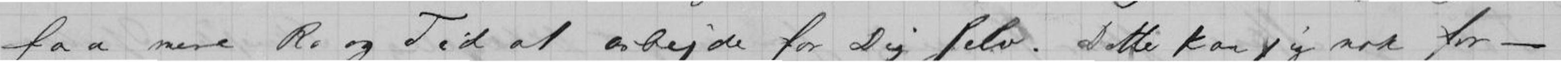faa mere Ro og Tid at arbejde for dig selv. Dette kan jeg nok for-
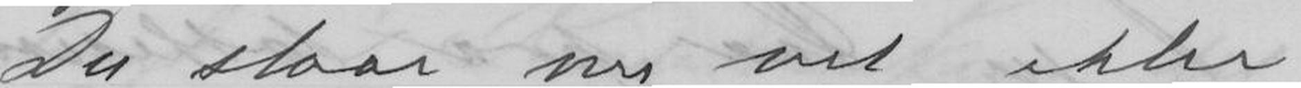Du slaar nu vel ikke
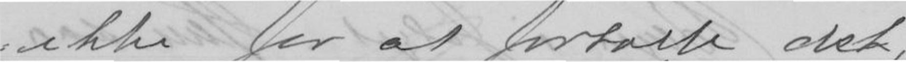ikke for at fortælle det,
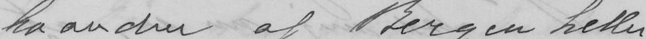haanden af Bergen heller,
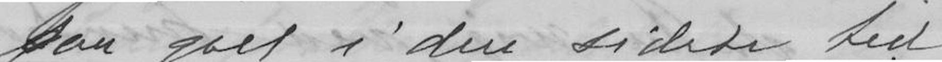for galt i den sidste tid,
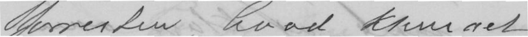forresten, hvad klimaet
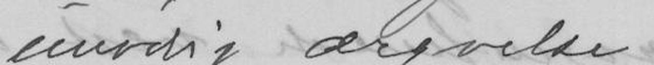unødig ærgrelse.
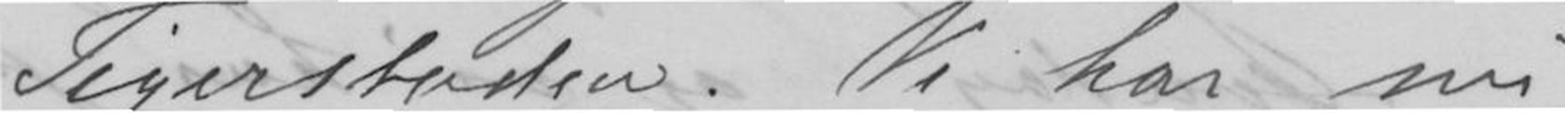Tigerstaden. Vi har nu
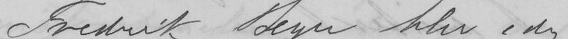Fredrik Beyer blir i dag
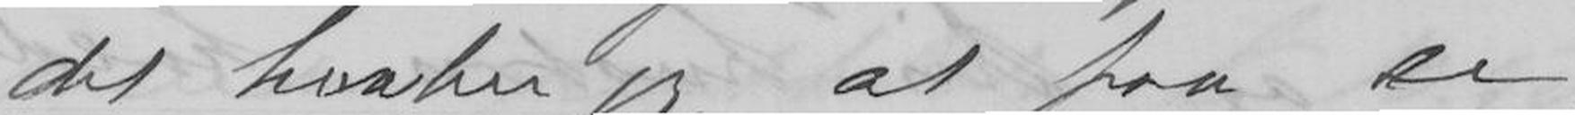det haaber jeg at faa se,
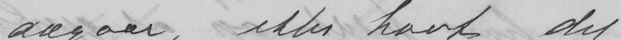angaar, ikke havt det
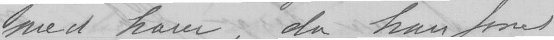med ham, da han først
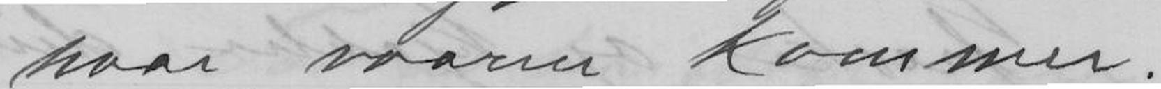naar vaaren kommer.
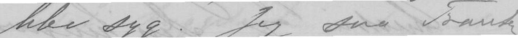blev syg. Jeg saa Frantz
